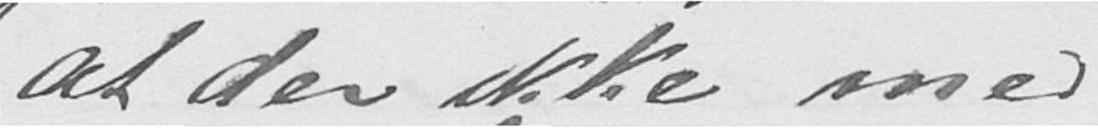at der ikke med
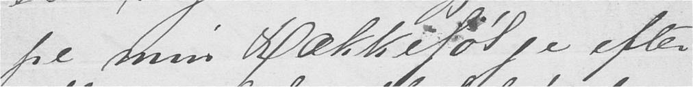pe min Rækkefølge efter
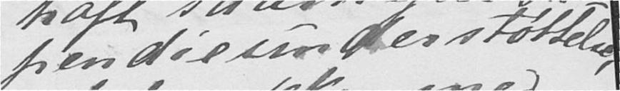pendieunderstøttelse,
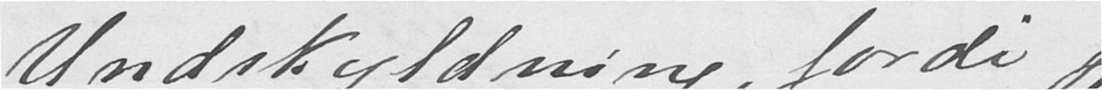Undskyldning, fordi jeg
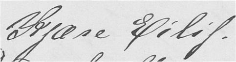Kjære Eilif.
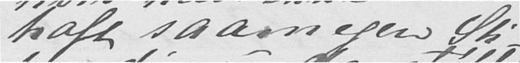haft saa megen Sti-
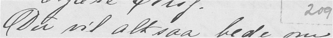Du vil altsaa bede om
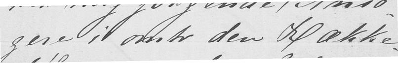gere i omtr den Række-
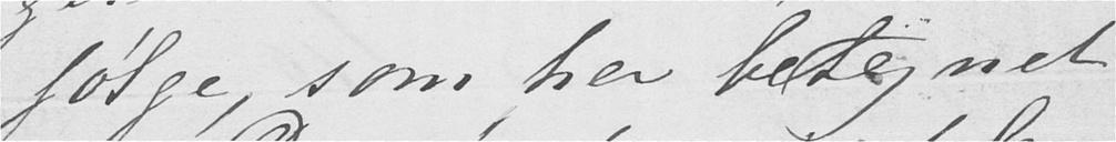følge, som her betegnet
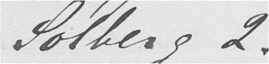Solberg 2.
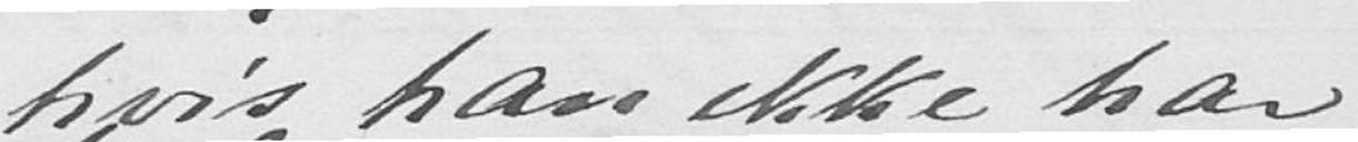hvis han ikke har
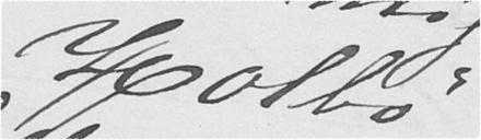Holbø 1
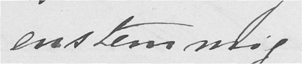enstemmig.
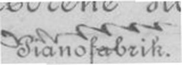Pianofabrik.
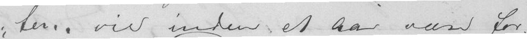ter, vil inden et Aar være for
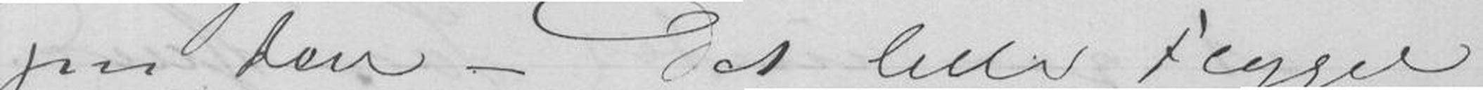paa den - Det lille Flygel
